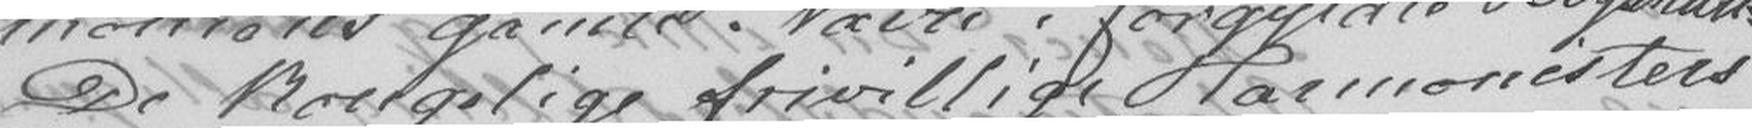De kongelige frivillige Harmonisters
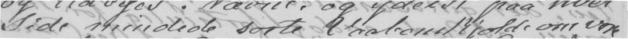Side mindede sorte Vaabenskjolde om vore
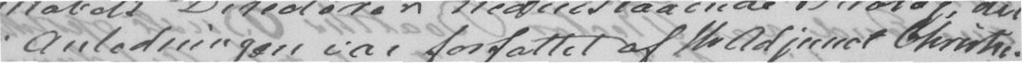i Anledningen var forfattet af Hr Adjunct Christie.
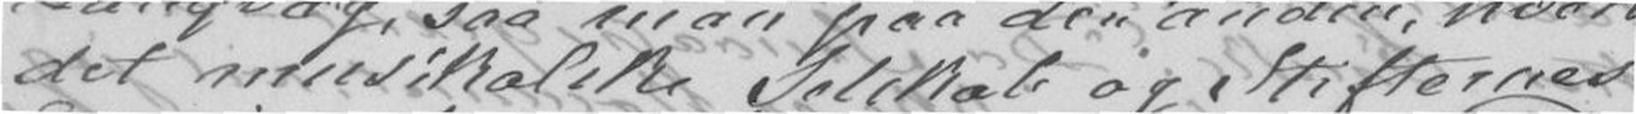det musikalske Selskab og Stifternes
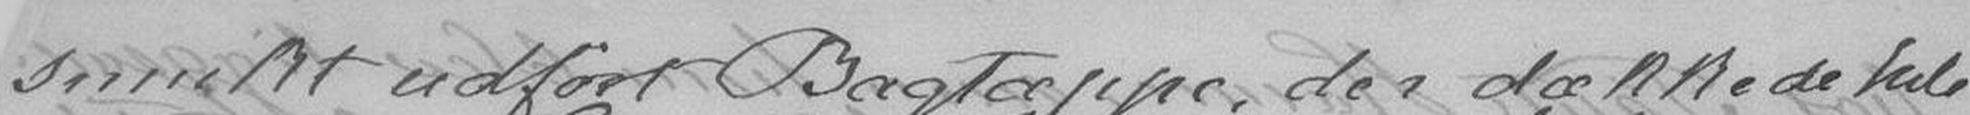smukt udført Bagtæppe, der dækkede hel
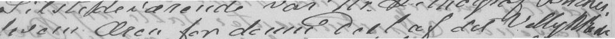hvem Æren for denne Deel af det Vellykkede
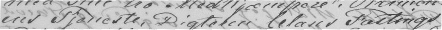ens Tjeneste, Digteren Claus Fastings
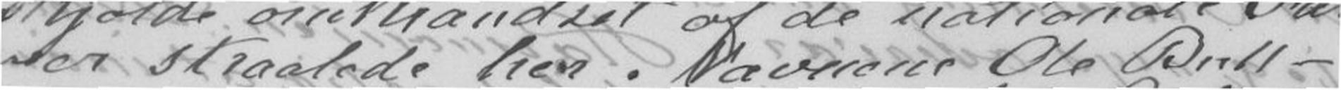ver straalede her Navnene Ole Bull -
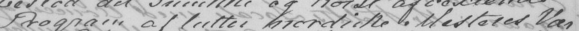Program af lutter nordiske Mesteres Vær-
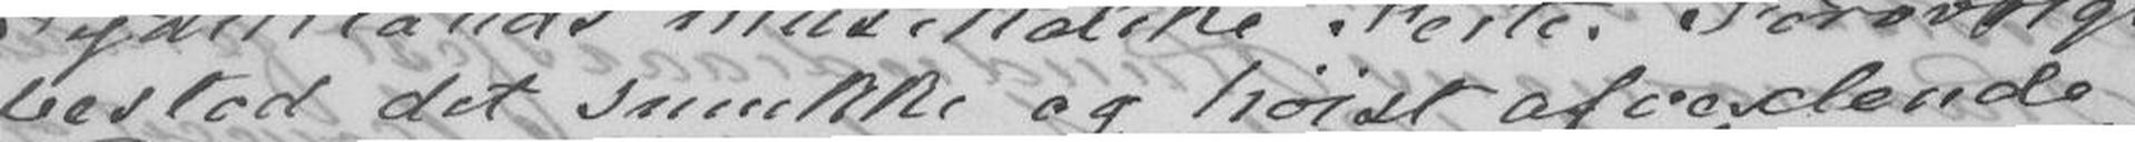bestod det smukke og høist afvexlende
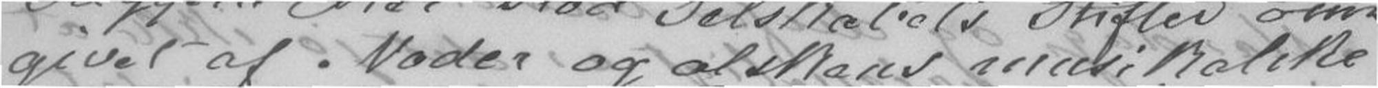givet af Noder og alskens musikalske
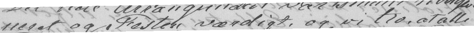neret og Festen værdigt, og vi tro, at alle
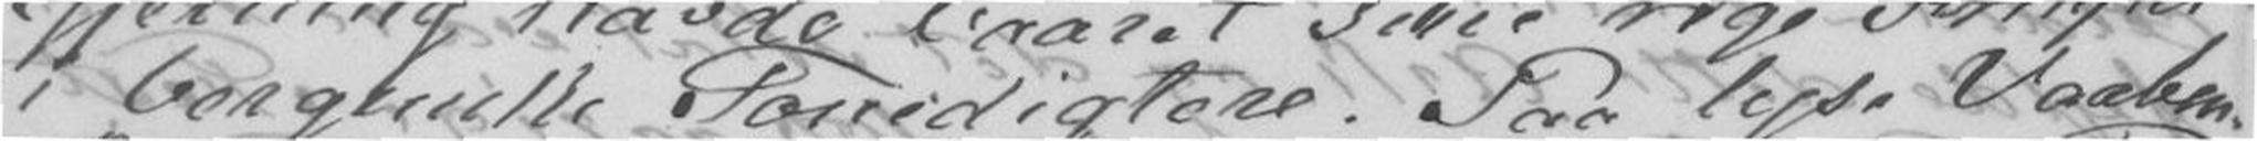i bergenske Tonedigtere. Paa lyse Vaaben-
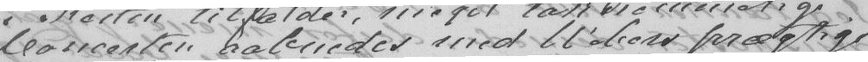Concerten aabnedes med Webers prægtige
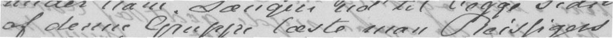af denne Gruppe læste man Reissigers
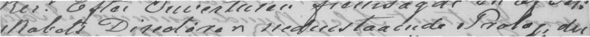skabets Directører nedenstaaende Prolog, der
